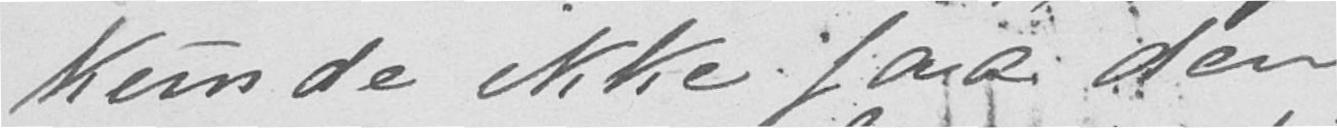kunde ikke faad den
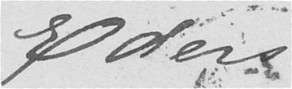Eders
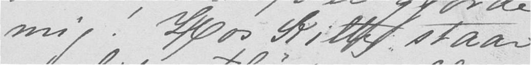mig! Hos Kitty staar
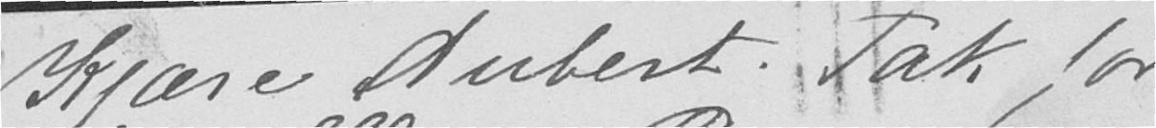Kjære Aubert. Tak for
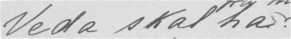Veda skal ha
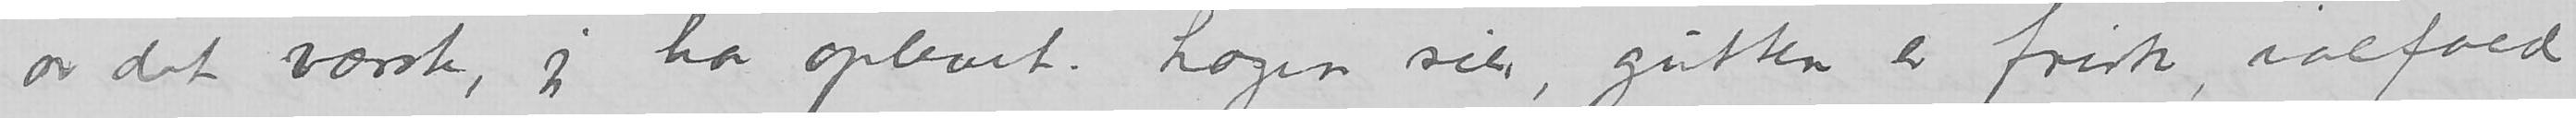av det værste, jeg har oplevet. Lægen sier, gutten er frisk, ialfald
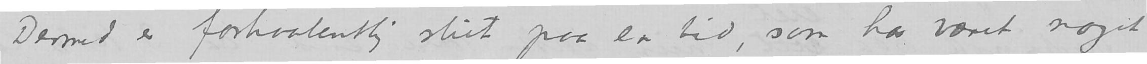Dermed er forhaabentlig slut paa en tid, som har været noget
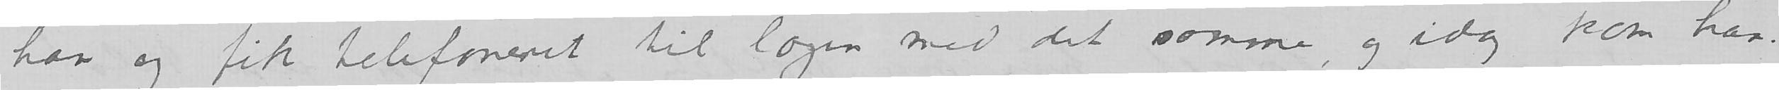han og fik telefonert til lægen med det samme, og idag kom han.
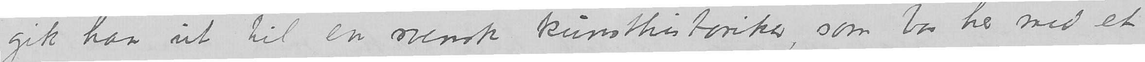gik han ut til en svensk kunsthistoriker, som bor her med et
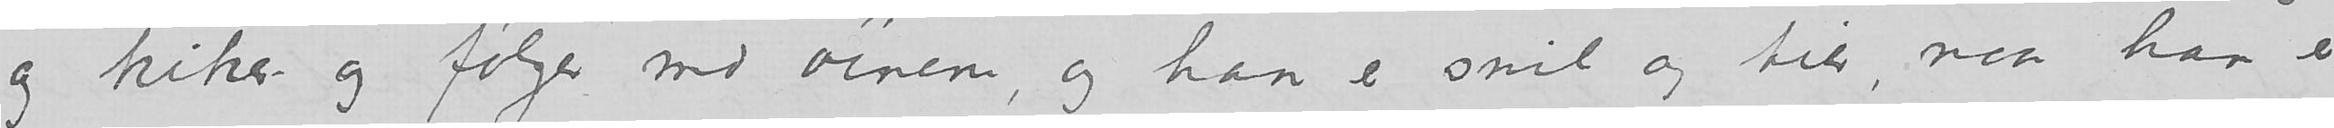og kiker og følger med øiene, og han er
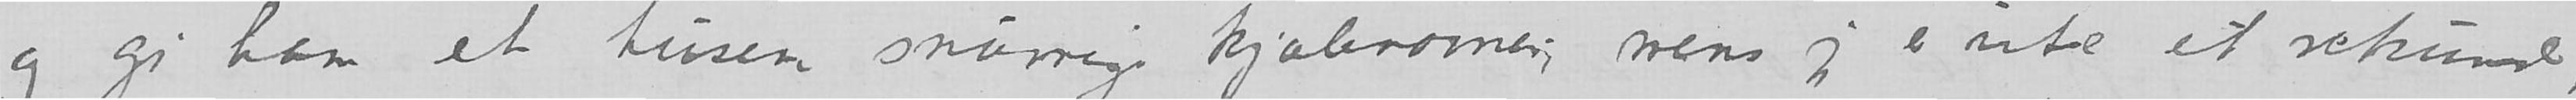og gi ham et tusen snurrige kjælenavner, mens jeg er ute et sekund,
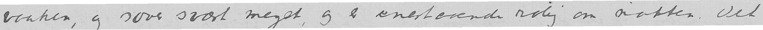snil og tier, naar han er vaaken, og sover svært meget, og er enestaaende rolig om natten. Det
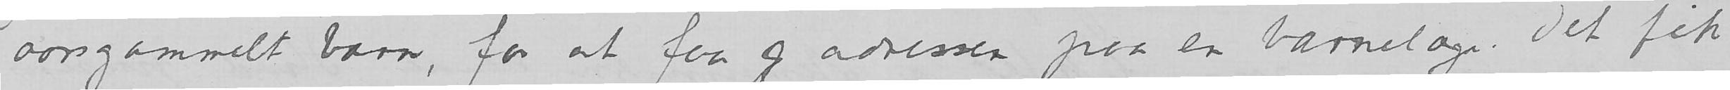aarsgammelt barn, for at faa g adressen paa en barnelæge. Det fik
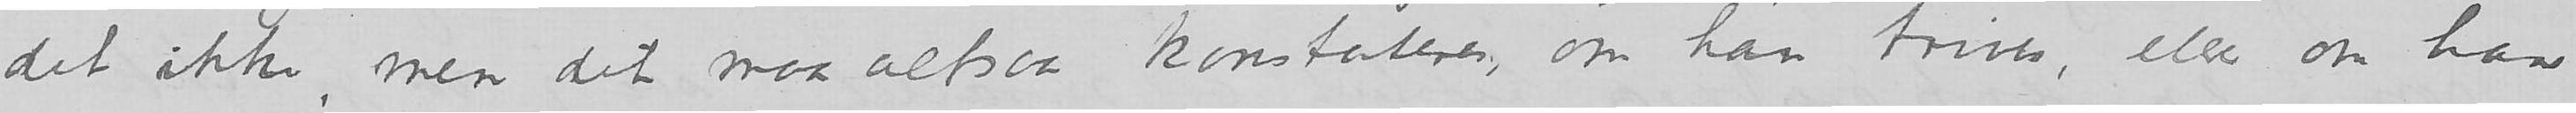det ikke, men det maa altsaa konstateres, om han
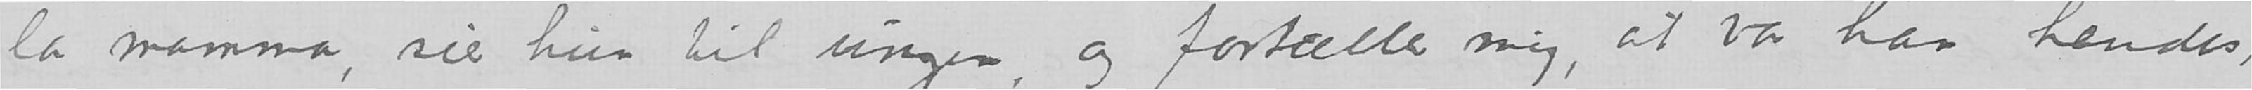la mamma, sier hun til ungen, og fortæller mig, at var han hendes,
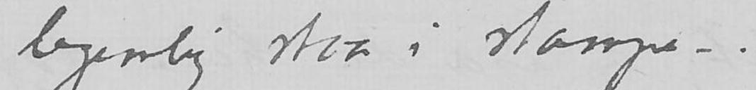trives, eller om han egentlig staar i stampe –.
